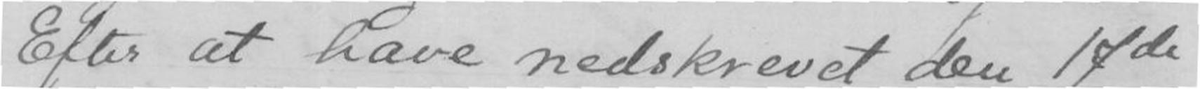Efter at have nedskrevet den 17de
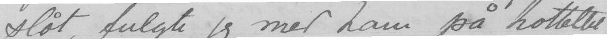slåt, fulgte jeg med ham på hottellet
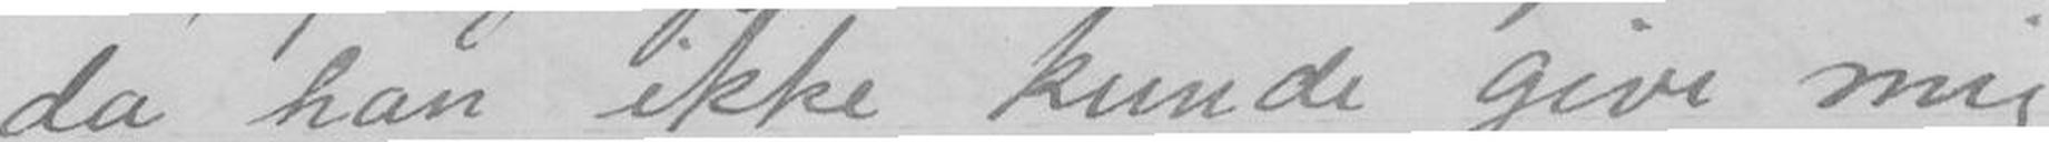da han ikke kunde give mig
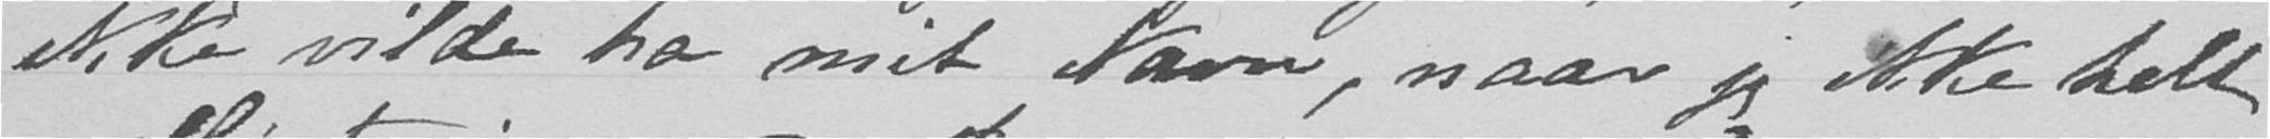ikke vilde ha mit Navn, naar jeg ikke helt
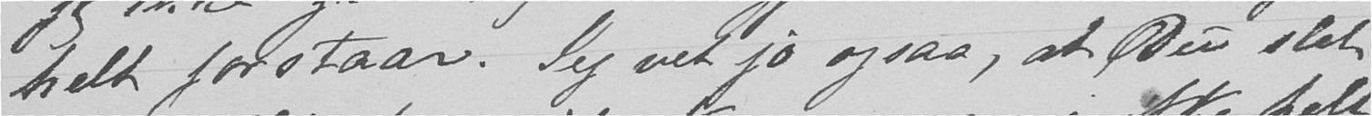helt forstaar. Jeg vet jo ogsaa, at Du slet
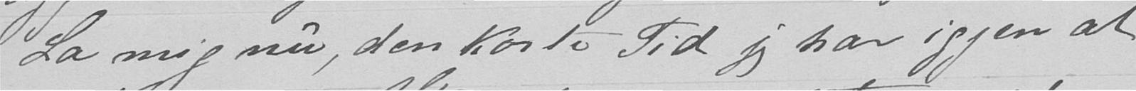La mig nu, den korte Tid jeg har igjen at
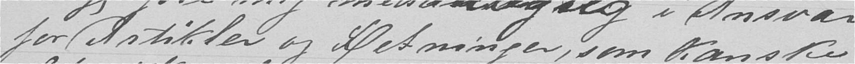for Artikler og Retninger, som kanske
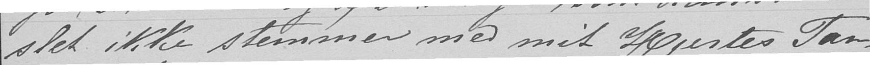slet ikke stemmer med mit Hjertes Tan-
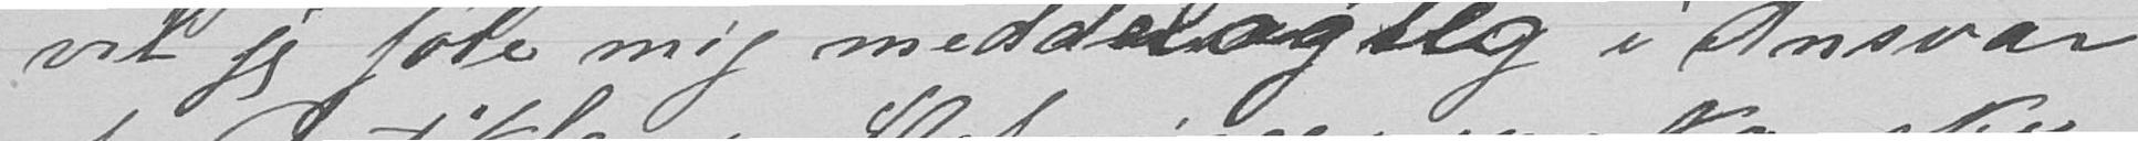vil jeg føle mig meddelagtlig i Ansvar
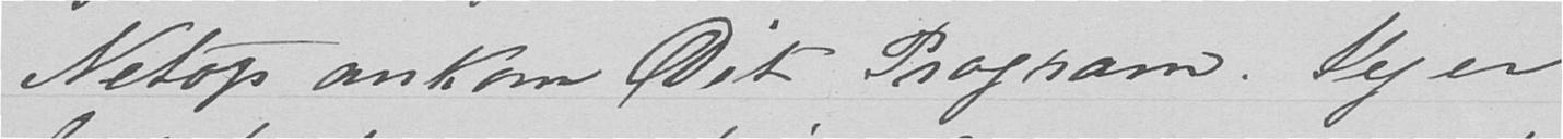Netop ankom Dit Program. Jeg er
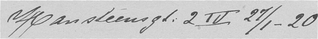Hansteensgt. 2 IV 27/1 - 20.
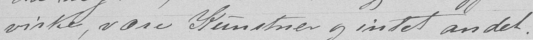virke, være Kunstner og intet andet.
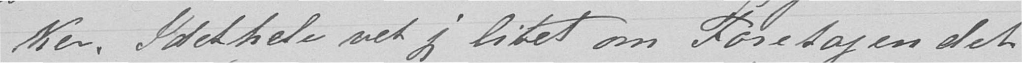ker. I det hele vet jeg litet om Foretagendet
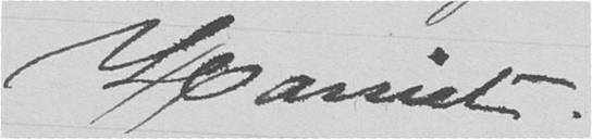Harriet
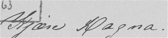Kjære Ragna.
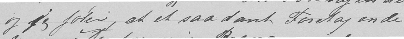og jeg føler, at et saadant Foretagende
